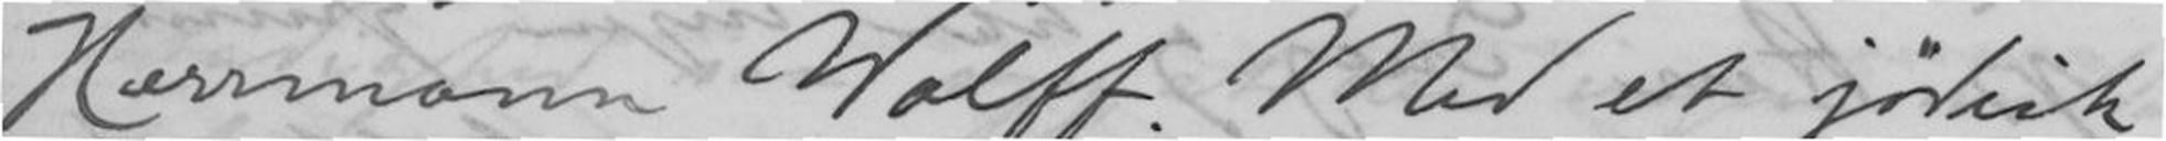Herrmann Wolff. Med et jødisk
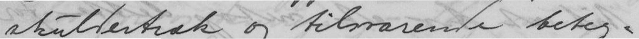skuldertræk og tilsvarende beteg-
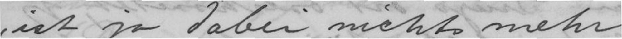ist ja dabei nichts mehr
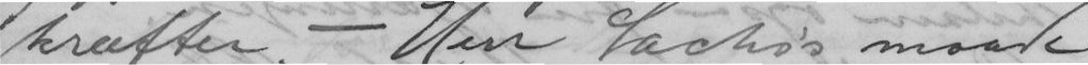kræfter. - Herr Sachs's maade
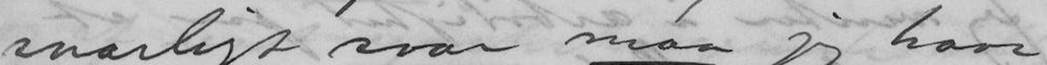snarligt svar maa jeg have
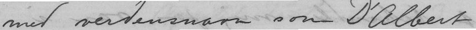med verdensnavn som D'Albert,
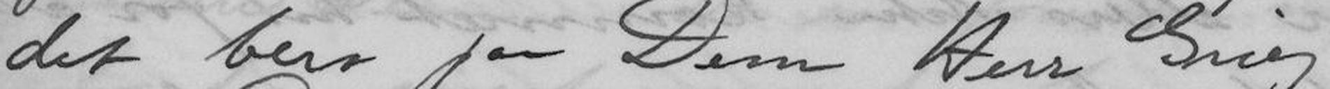det bero paa Dem Herr Grieg
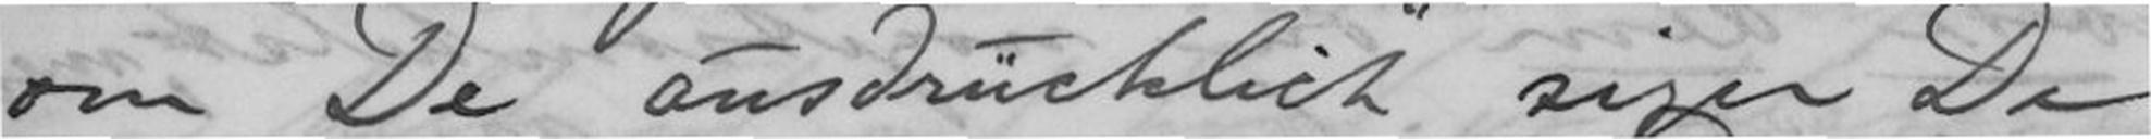om De "ausdrücklich" siger De
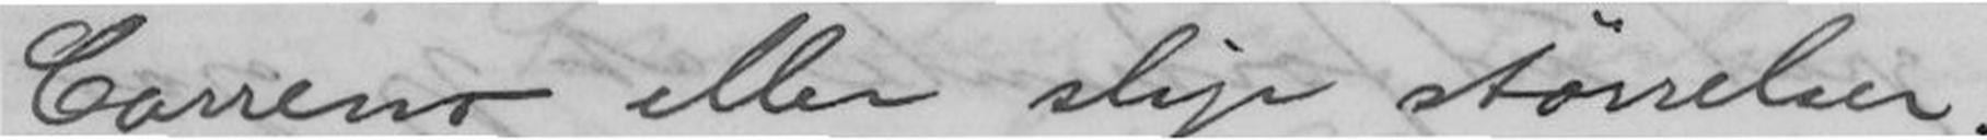Carreno eller slige størrelser.
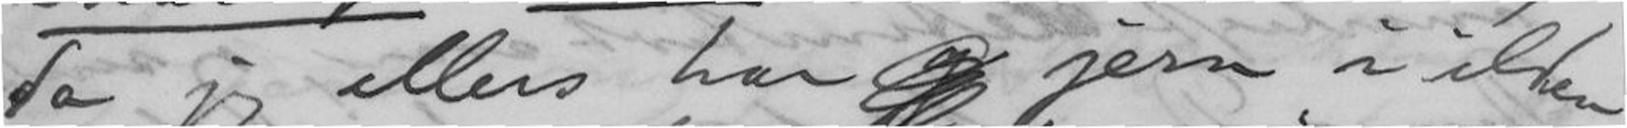da jeg ellers har jern i ilden
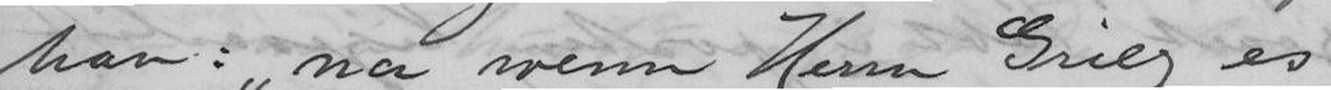han: "na wenn Herrn Grieg es
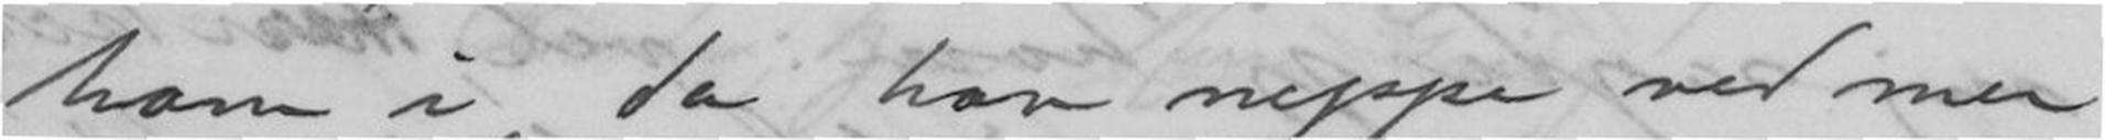ham i, da han neppe ved mer
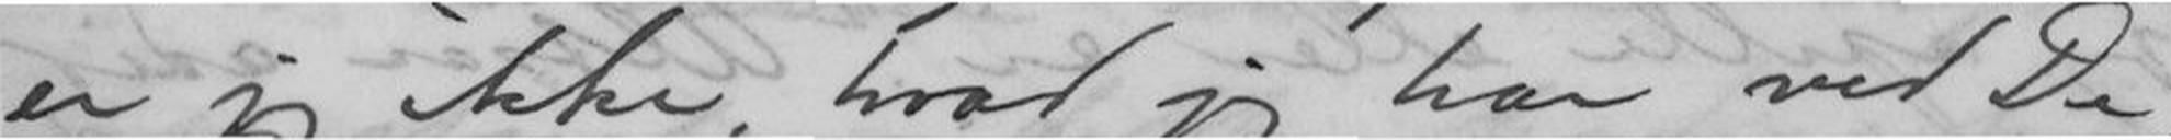er jeg ikke, hvad jeg har ved De,
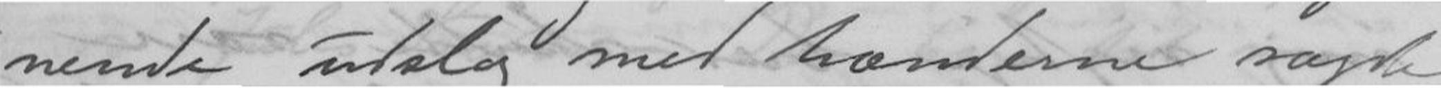nende udslag med hænderne sagde
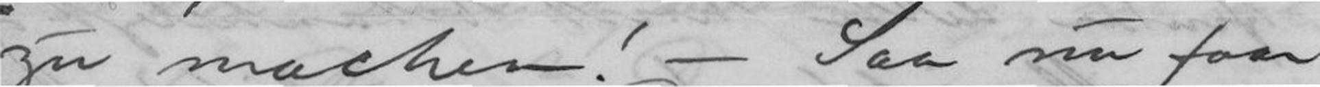zu machen! - Saa nu faar
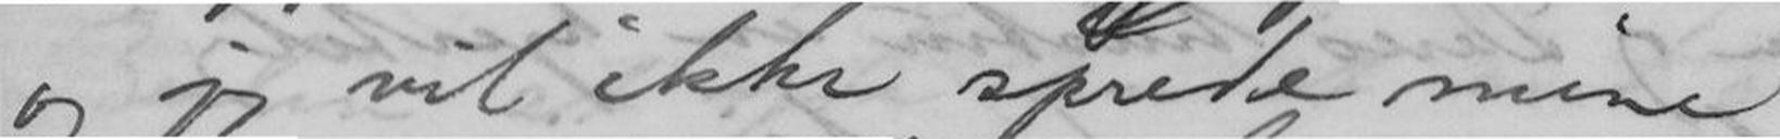og jeg vil ikke sprede mine
